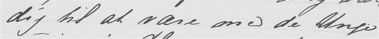dig til at være med de Unge
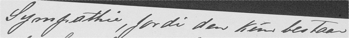Sympathie, fordi den kun bestaar
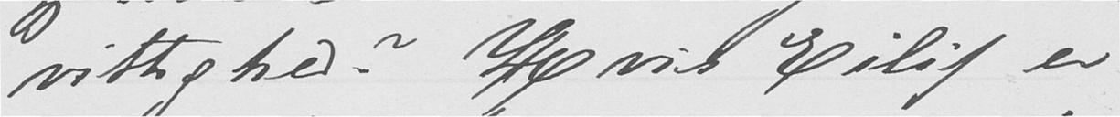vittighed? Hvis Eilif er
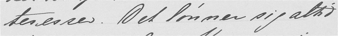teresser. Det fønner sig altid
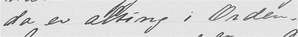da er alting i Orden.
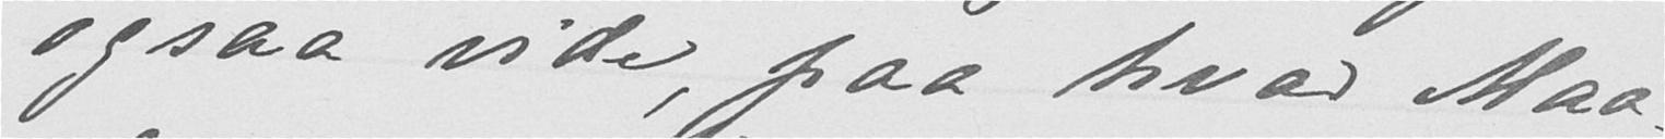ogsaa vide paa hvad Maa-
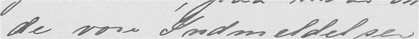de vore Indmeldelser
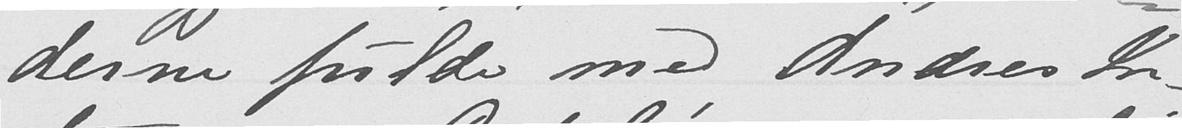derne fulde med Andres In-
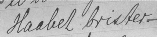Haabet brister - - -
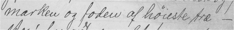marken og foden af høieste træ -
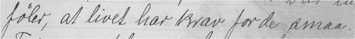- føler, at livet har krav for de smaa.
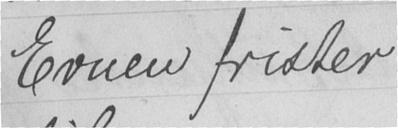Evnen frister
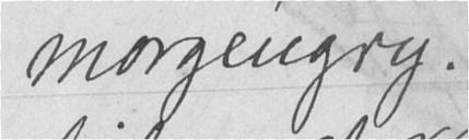morgengry.
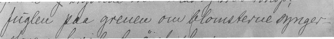fuglen paa grenen om blomsterne synger -
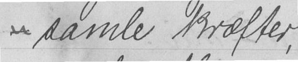samle kræfter,
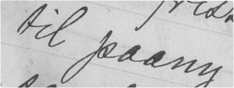til paany
